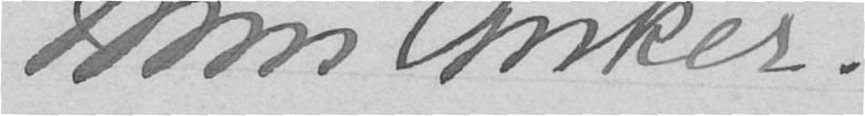Nini Anker
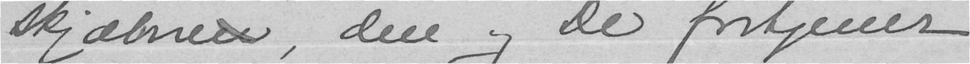skjæbne, den og de fortjener.
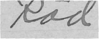Rød
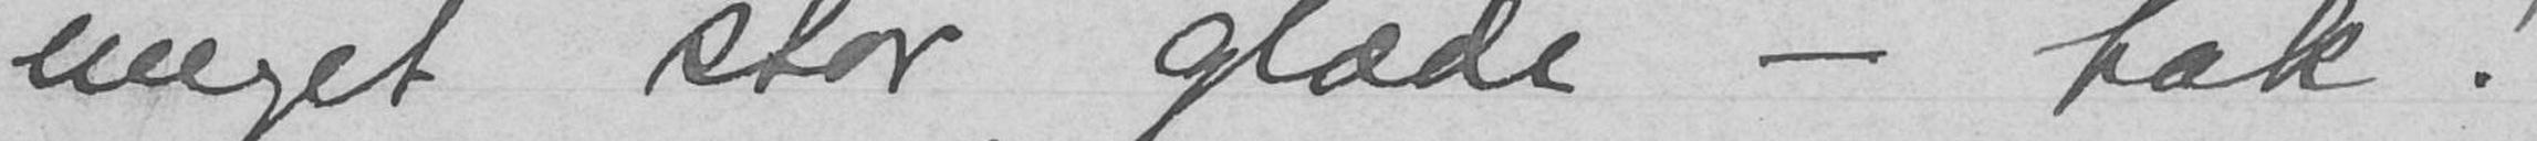meget stor glæde - tak!
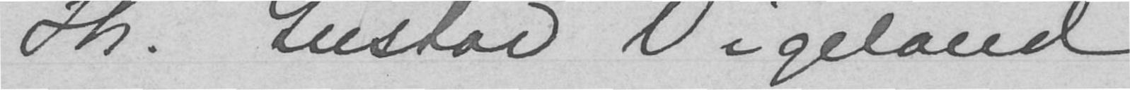Hr. Gustav Vigeland
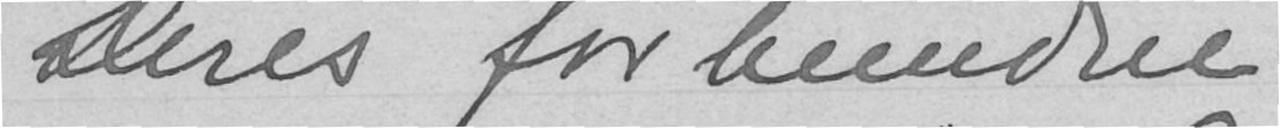Deres forbundne
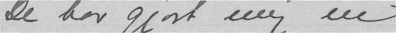De har gjort mig en
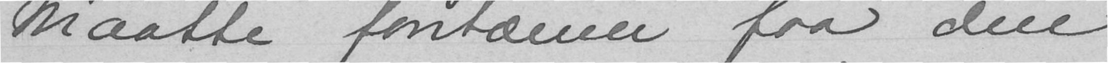Maatte fontænen faa den
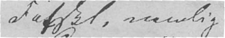Falskt, nemlig
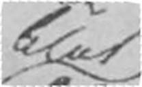blot
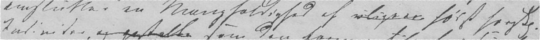omslutter en Mangfoldighed af uligear høist forskj.
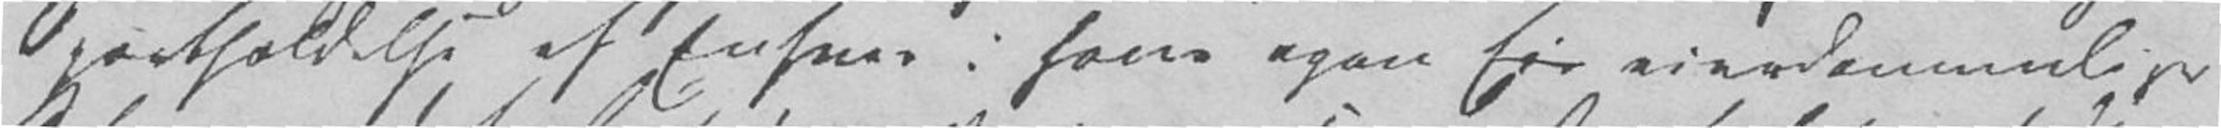Opretholdelse af Enhver i hans egen Eie eiendommelige
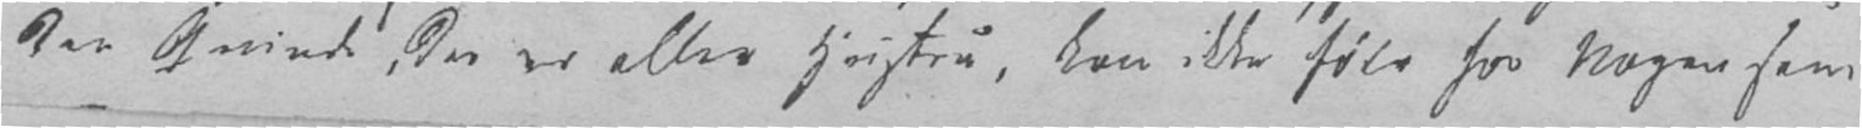den Qvinde, der er alles Hustru, kan ikke føle for Nogen som
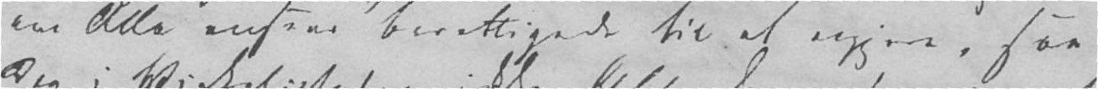om Alle ansees berettigede til at afgjøre, saa
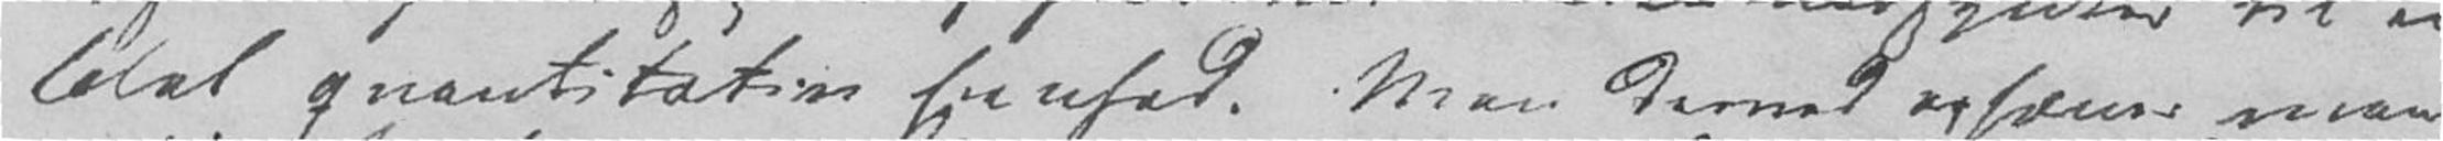blot qvantitativ Eenhed. Men derved ophæver man
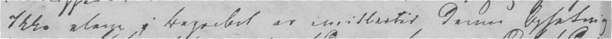Ikke alene i Begrebet er imidlertid denne Opfatning
